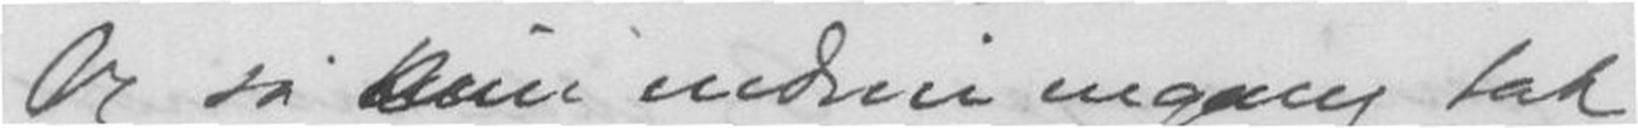Og så kun endnu engang tak
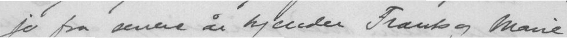jo fra senere år kjender Frants og Marie
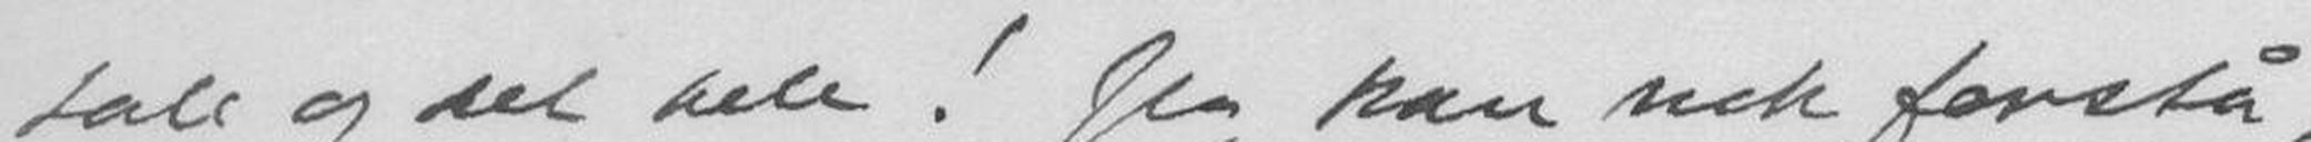tale og det hele! Jeg kan nok forstå,
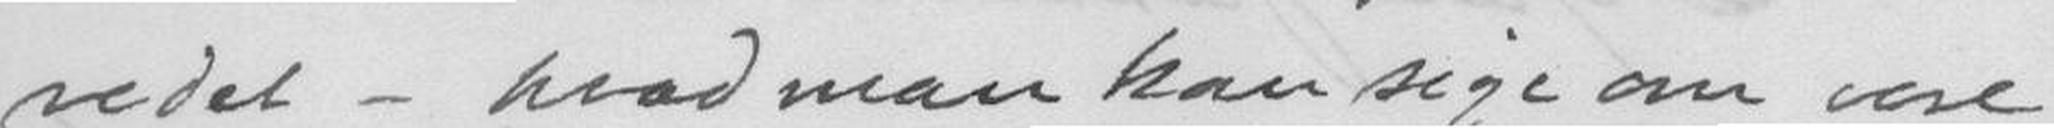redet - hvad man kan sige om vore
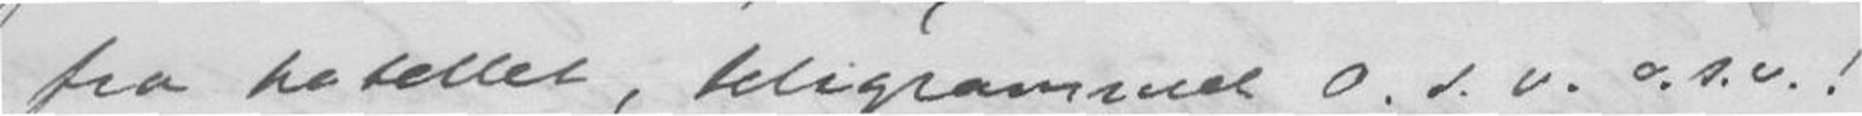fra hotellet, telegrammet o.s.v. o.s.v.!
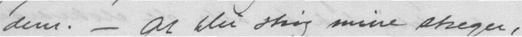dem. - At Du strøg mine streger,
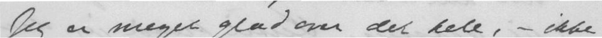Jeg er meget glad over det hele, - ikke
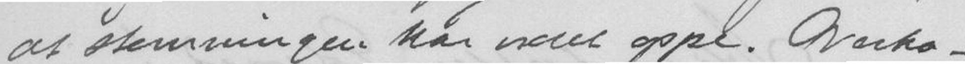at stemningen har været oppe. Overko-
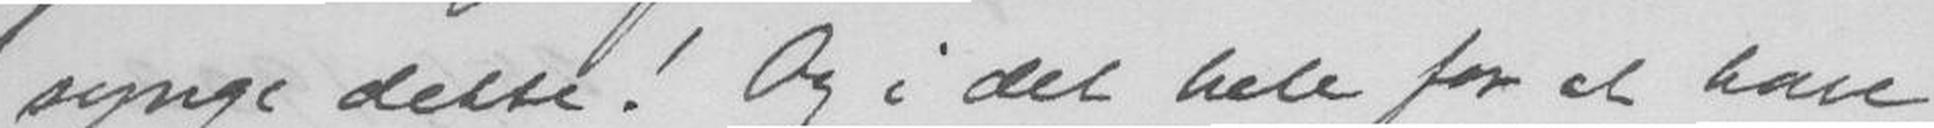synge dette! Og i det hele for at bare
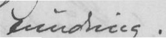erindring.
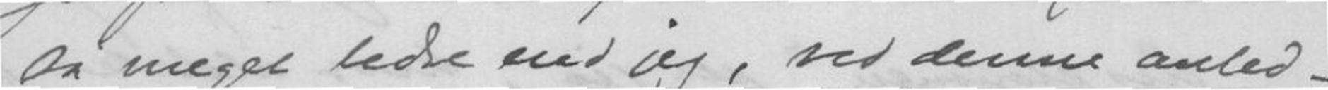så meget bedre end jeg, ved denne anle-
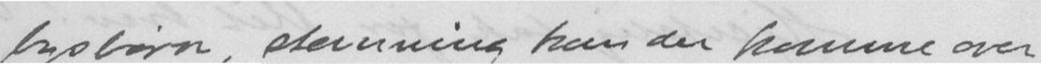bysbørn, stemning kan der komme over
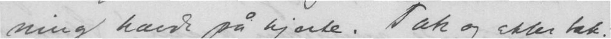dning havde på hjerte. Tak og atter tak!
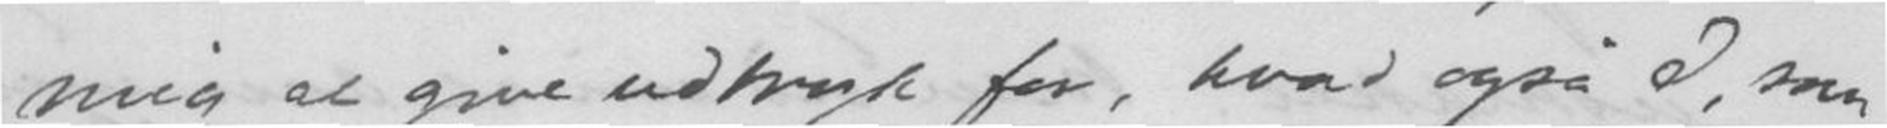mig at give udtryk for, hvad også I, som
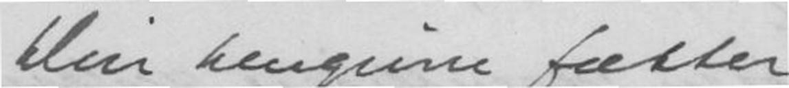Din hengivne fætter
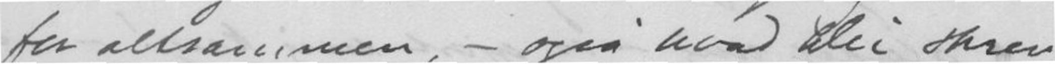for altsammen, - også hvad Du skrev
